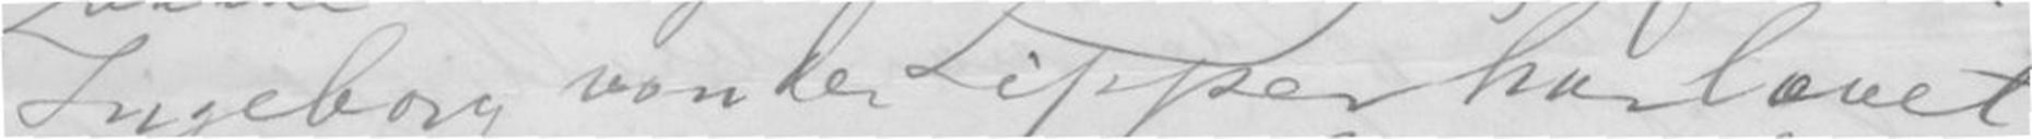Ingeborg von der Lipper har lovet
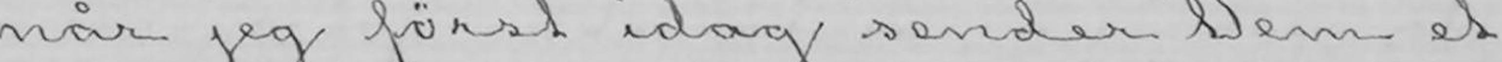når jeg først idag sender Dem et
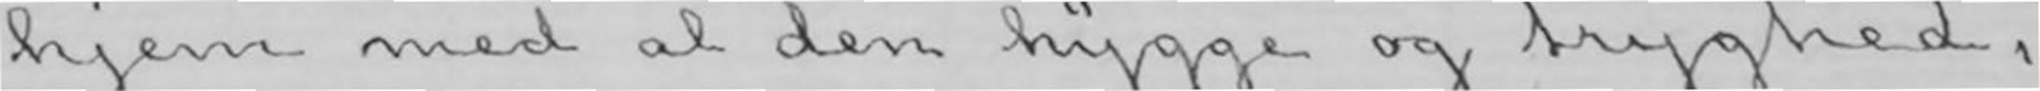hjem med al den hygge og tryghed,
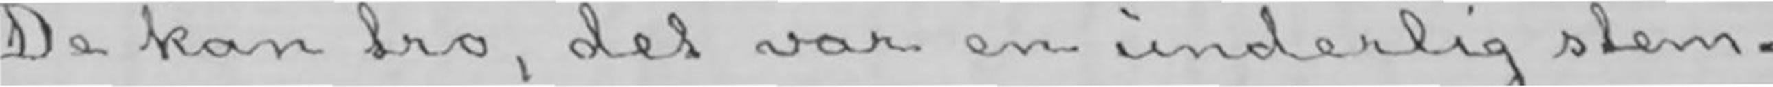De kan tro, det var en underlig stem-
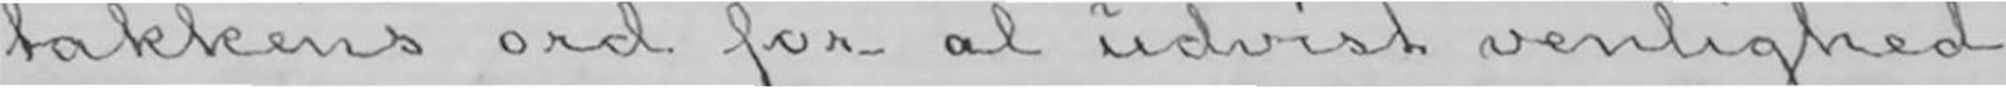takkens ord for al udvist venlighed
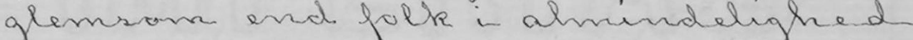glemsom end folk i almindelighed
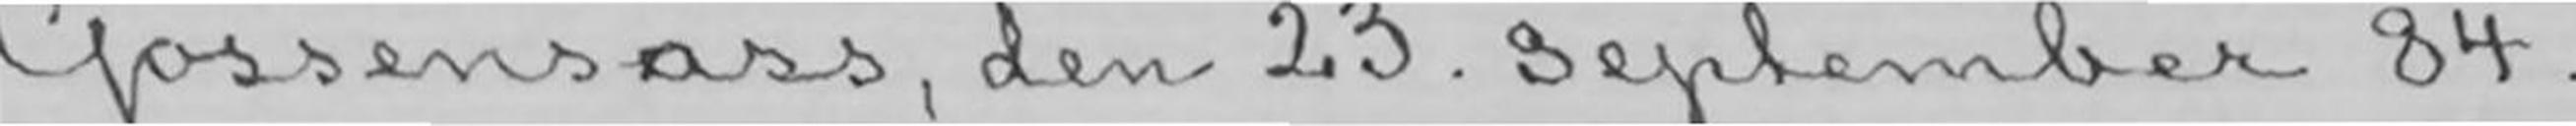Gossensass, den 23. september 84.
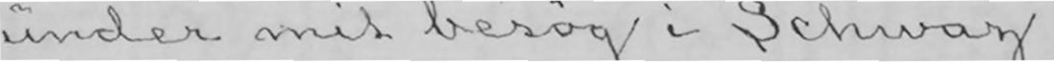under mit besøg i Schwaz.
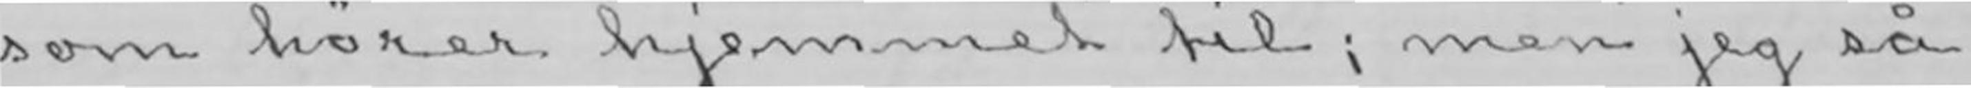som hører hjemmet til; men jeg så
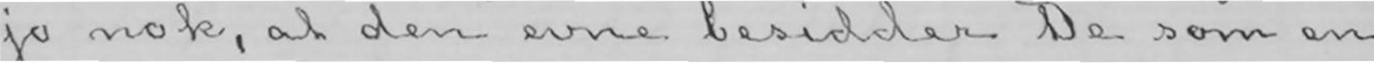jo nok, at den evne besidder De som en
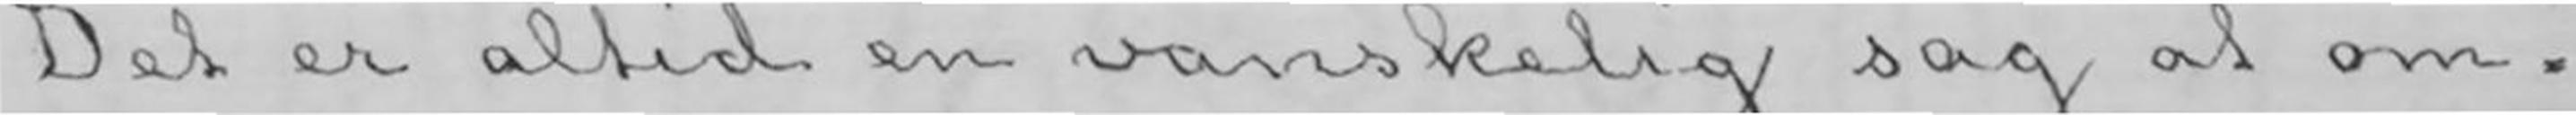Det er altid en vanskelig sag at om-
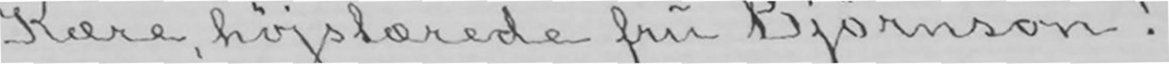Kære, højstærede fru Bjørnson!
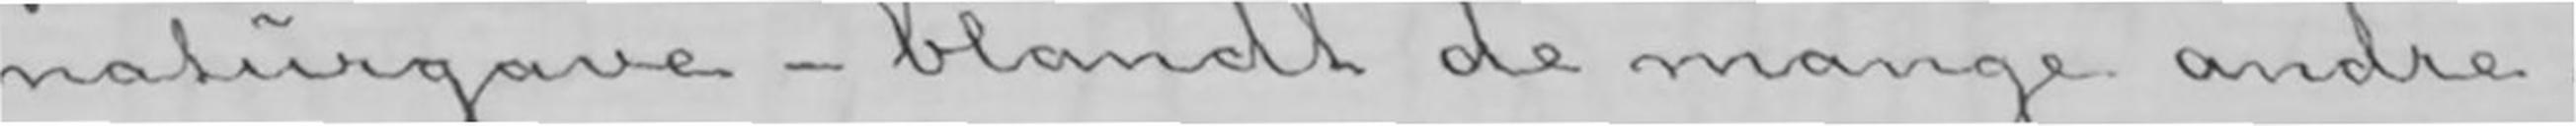naturgave - blandt de mange andre.
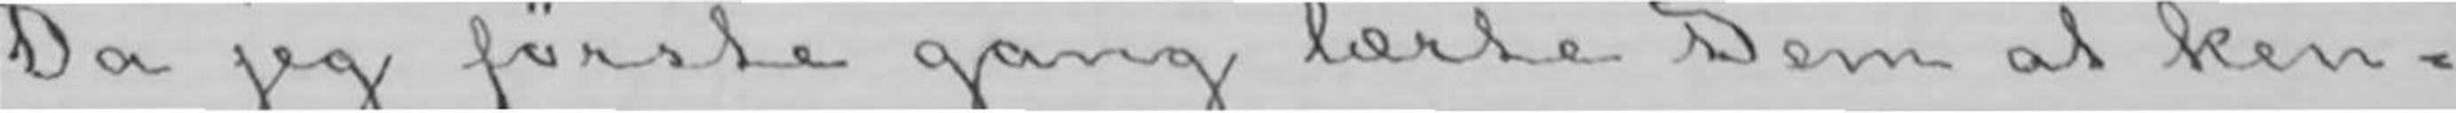Da jeg første gang lærte Dem at ken-
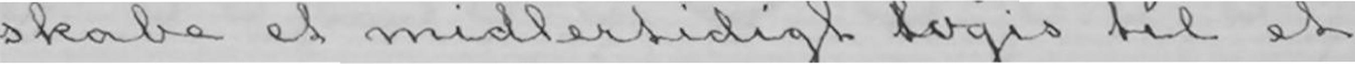skabe et midlertidigt tilogis til et
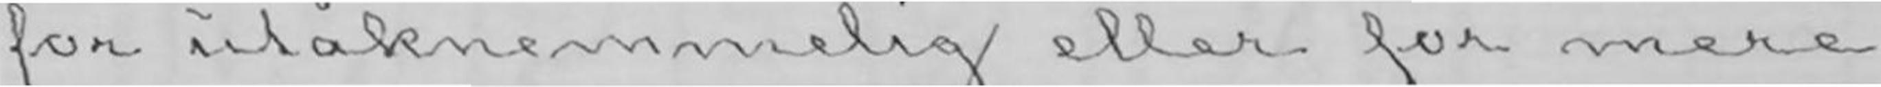for utaknemmelig eller for mere
